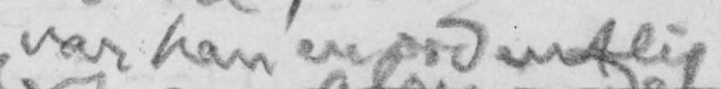var han en ordentlig
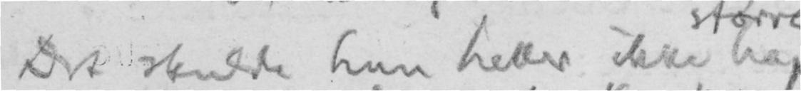Det skulde hun heller ikke ha
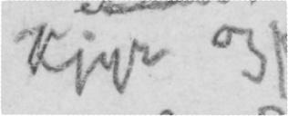Kjyr og
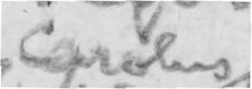Carolus
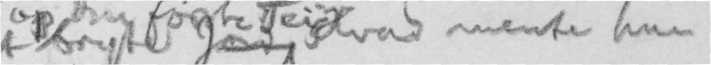op den første Teig. Hvad mente hun
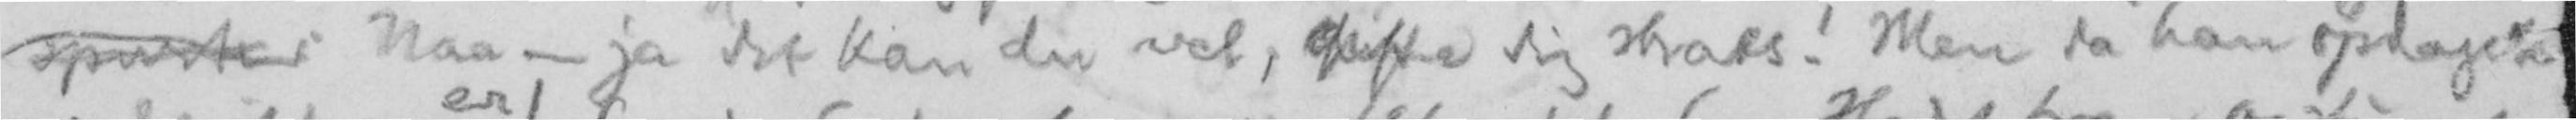spurte: Naa - ja det kan du vel, gifte dig straks! Men da han opdagede
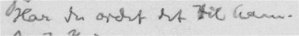Har du ordet det til ham.
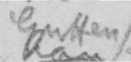Gutten
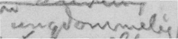ungdommelig
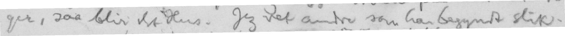ger, saa blir det Hus. Jeg vet andre som har begyndt slik.
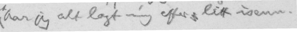har jeg alt lagt mig efter , litt isenn.
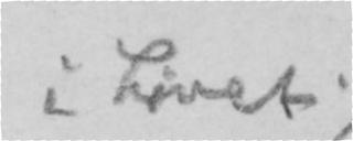i Livet.
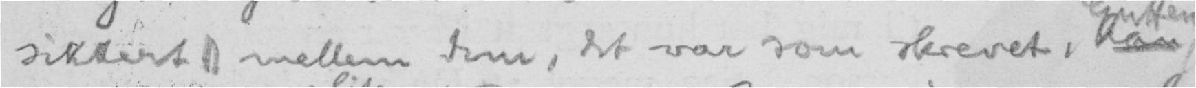sikkert || mellem dem, det var som skrevet, han
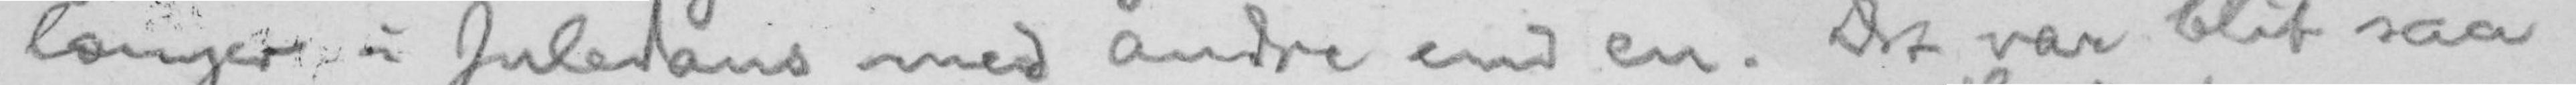længer i Juledans med andre end en. Det var blit saa
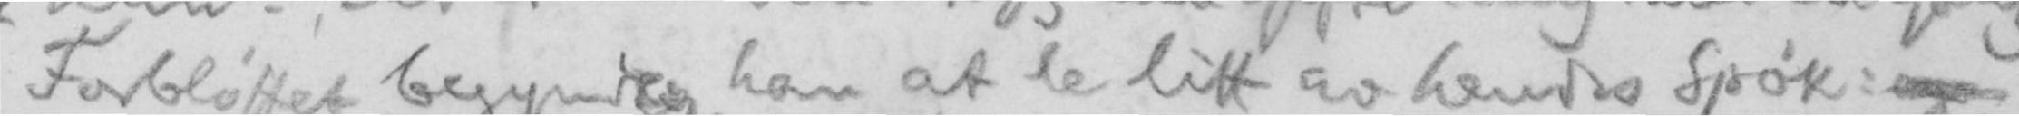Forbløffet begynder han at le litt av hendes Spøk: og
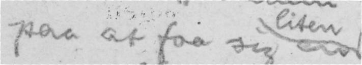paa at faa sig en
